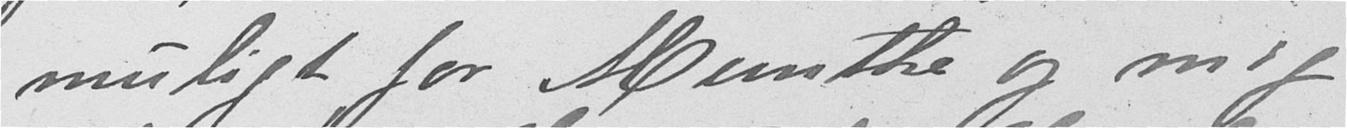muligt for Munthe og mig
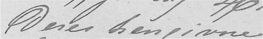Deres hengivne
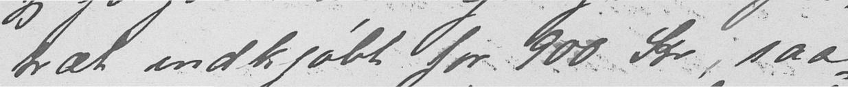træt indkjøbt før 900 kr, saa-
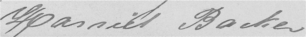Harriet Backer
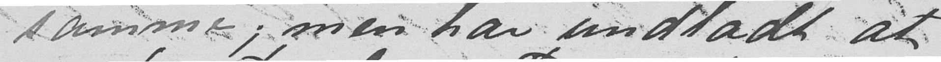samme; men har undladt at
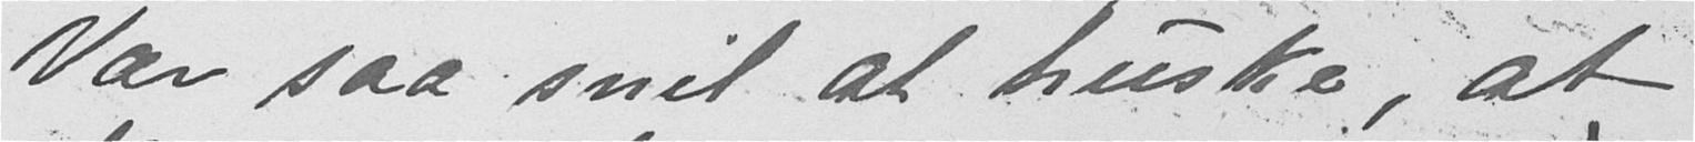Vær saa snil at huske, at
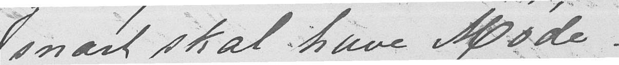snart skal have Møde?
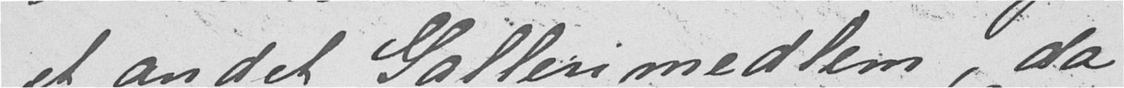et andet Gallerimedlem, da
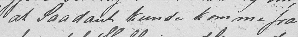at Saadant kunde komme fra
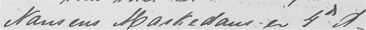Nansens Maskedans er 4de A-
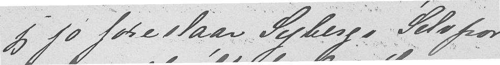jeg jo foreslaar Sybergs Selvpor-
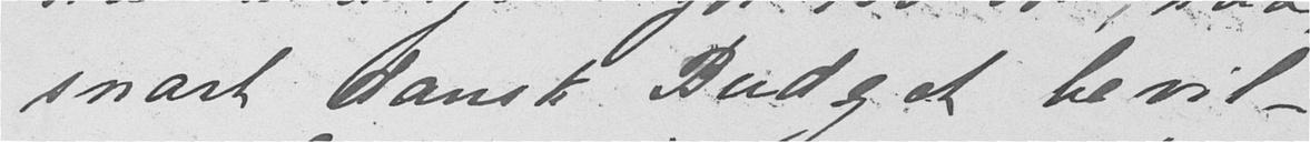snart dansk Budget bevil-
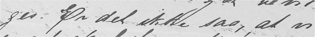ges. Er det ikke saa, at vi
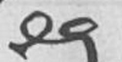eg
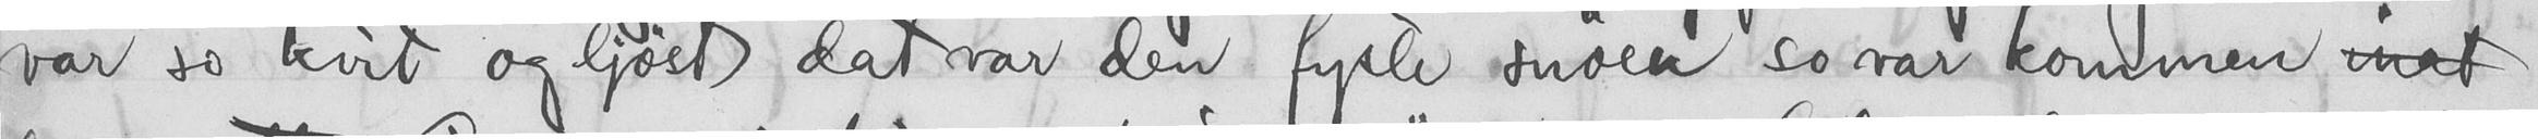var so kvit og ljøst, dat var den fyrte snøen so var kommen
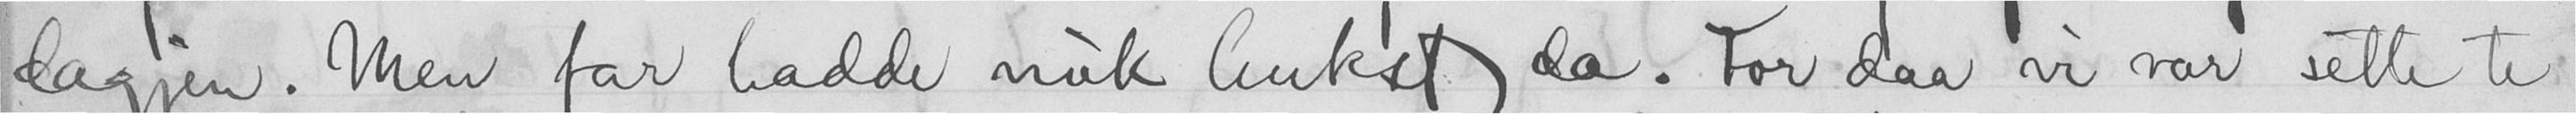dagjen. Men far hadde nuk hukst da. For daa vi var sette te
